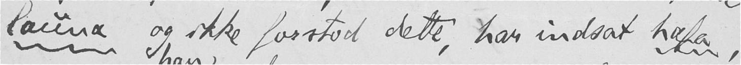launa og ikke forstod dette, har indsat hafa,
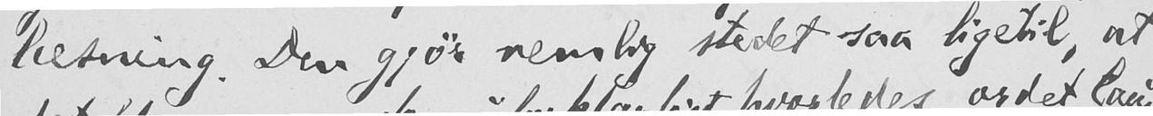læsning. Den gjør nemlig stedet saa ligetil, at
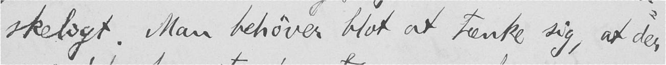skeligt. Man behøver blot at tænke sig, at der
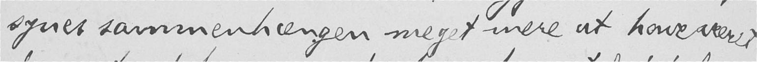synes sammenhængen meget mere at have vært
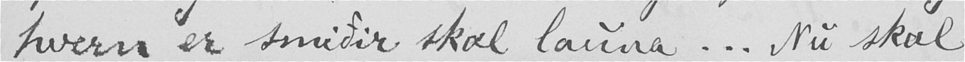hvem er smiðir skal launa... Nu skal
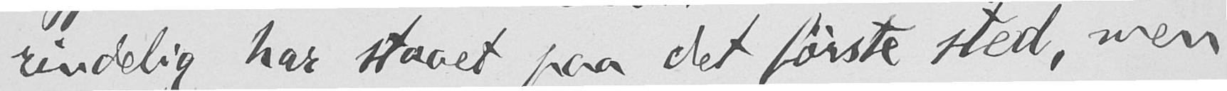rindelig har staaet paa det første sted, men
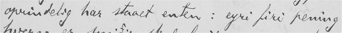oprindelig har staaet enten: egri firi pening
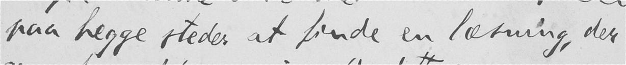paa begge steder at finde en læsning, der
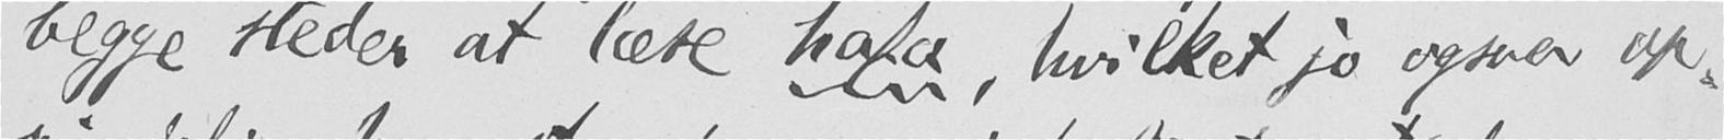begge steder at læse hafa, hvilket jo ogsaa op-
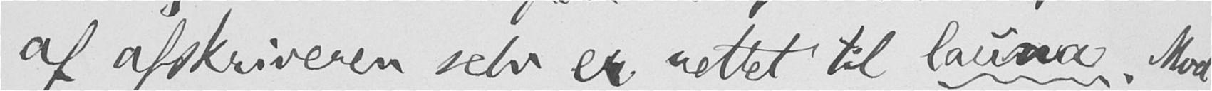af afskriveren selv er rettet til launa. Mod
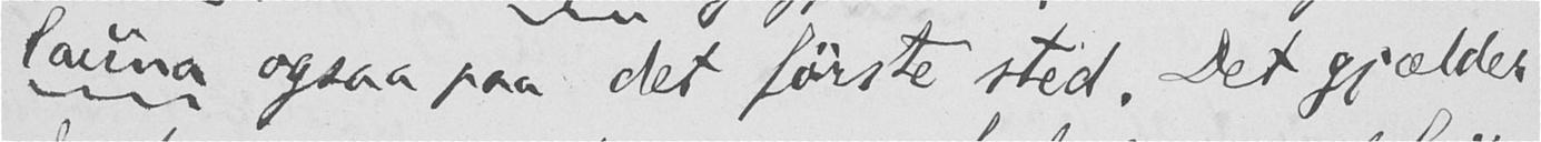launa ogsaa paa det første sted. Det gjælder
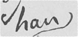han
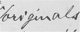originals
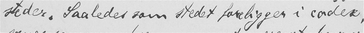steder. Saaledes som stedet foreligger i codex,
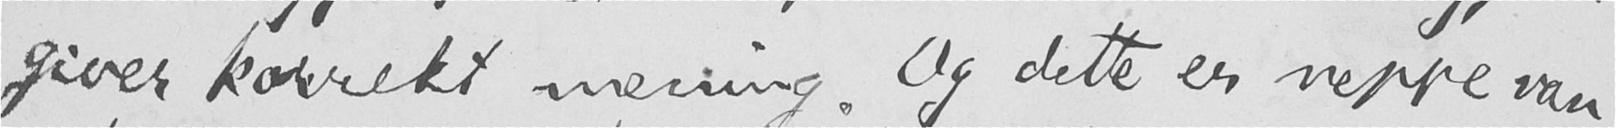giver korrekt mening. Og dette er neppe van-
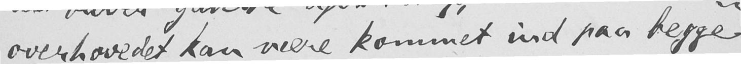overhovedet kan være kommet ind paa begge
